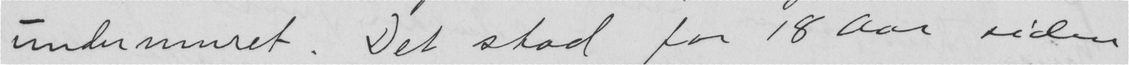undermuret. Det stod for 18 Aar siden
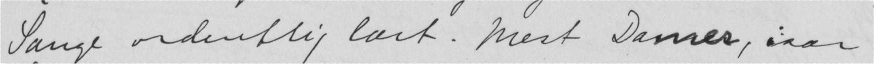Sange ordentlig lært. Mest Damer, iaar
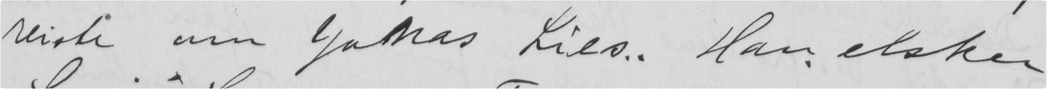reiste om Jonas Lies. Han elsker
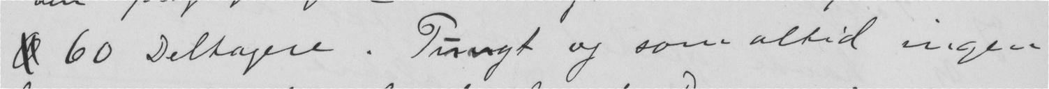60 Deltagere. Tungt og som altid ingen
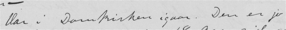Var i Domkirken igaar. Den er jo
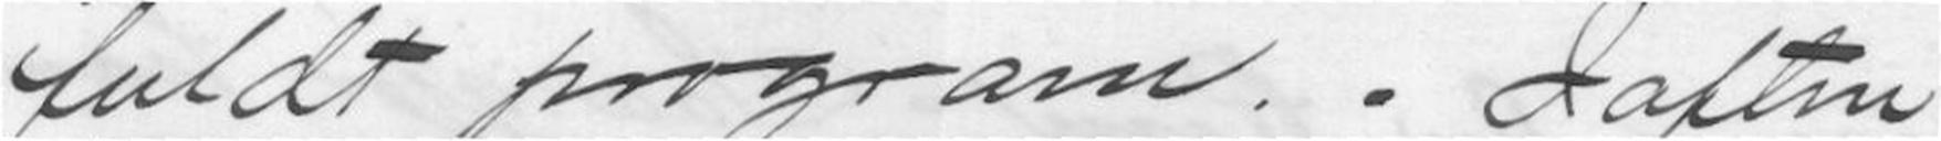fuldt program. - Iaften
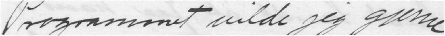Programmet vilde jeg gjerne
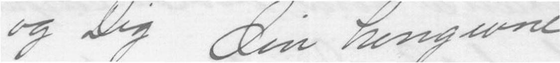og Dig din hengivne
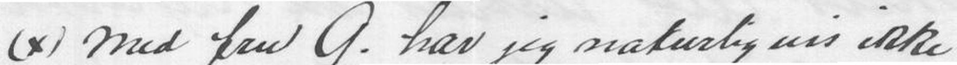(x) Med fru G. har jeg naturligvis ikke
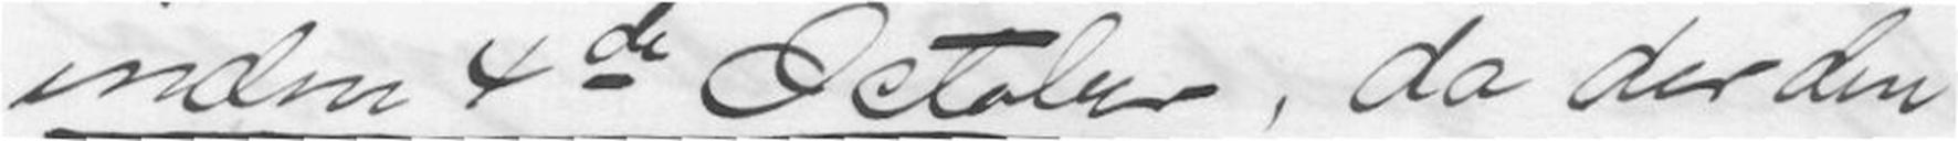inden 4de October, da der den
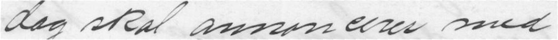dag skal annonceres med
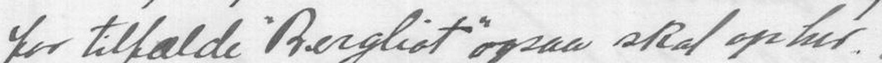for tilfælde "Bergliot" ogsaa skal op her.
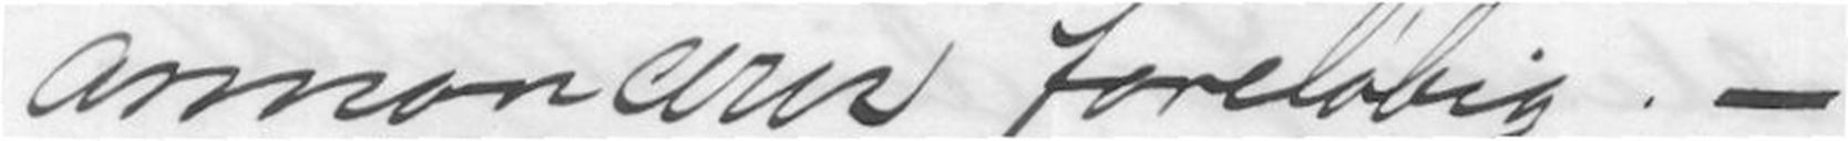annonceres foreløbig. -
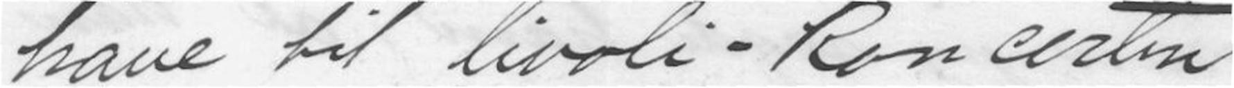have til tivoli - koncerten
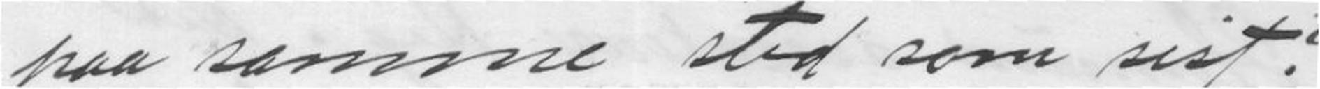paa samme sted som sist?
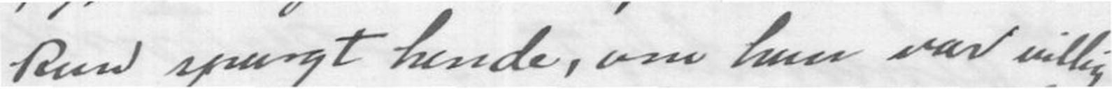kun spurgt hende, om hun var villig
